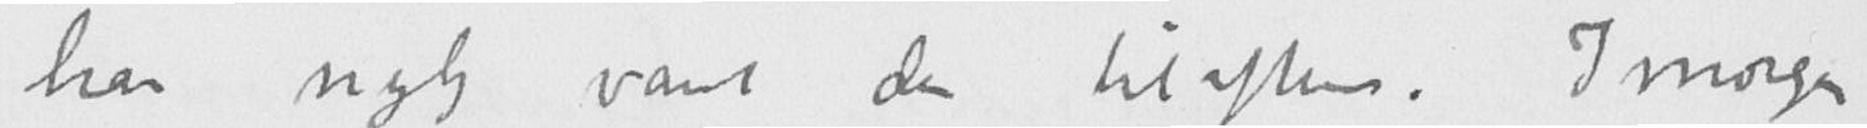har nylig været der tilaftens. I morgen
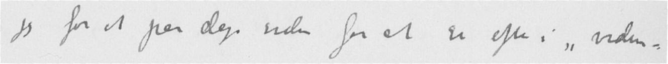jeg for et par dager siden for at se efter i "viden-
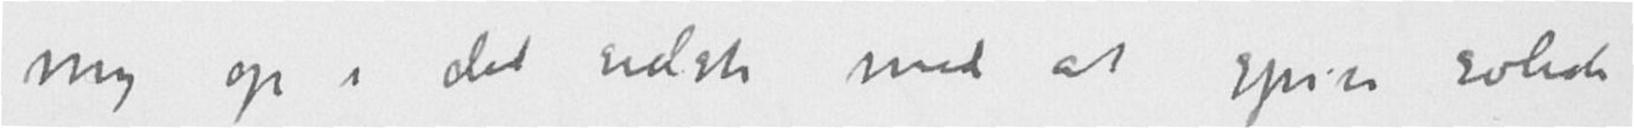mig op i det sidste med at spise solide
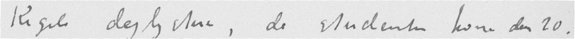Kegels dagligstue, da studenten kom den 20.
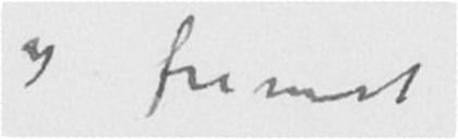og fremst
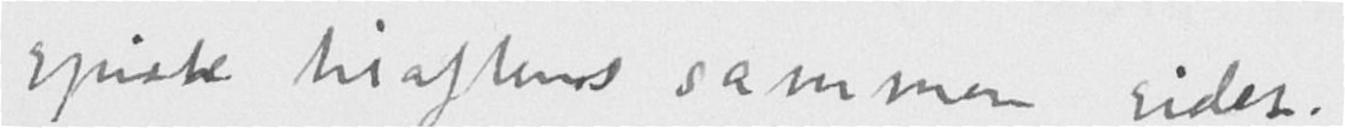spiste tilaftens sammen sidst.
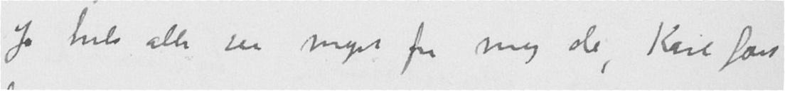Jo hils alle saa meget fra mig da, Karl først
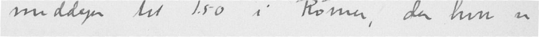middager til 1.50 i Roma, der hvor vi
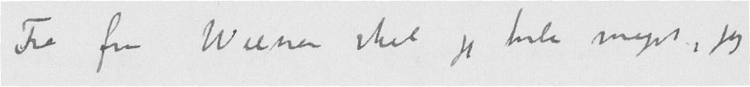Fra fru Wiener skal jeg hilse meget, jeg
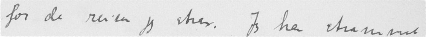for da reiser jeg strax. Jeg har strammet
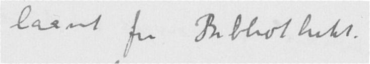laant fra Bibliotheket.
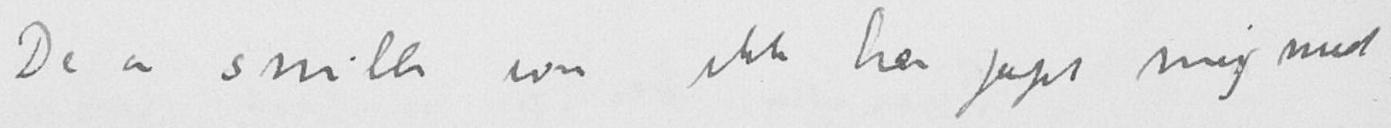De er snille som ikke har jaget mig midt
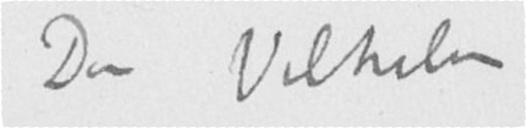Din Vilhelm
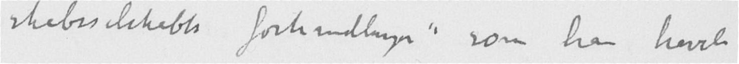skabsselskabets forhandlinger" som han havde
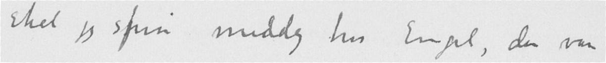skal jeg spise middag hos Engel, der var
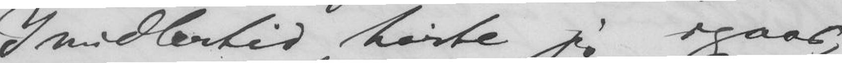Imidlertid hørte jeg ogsaa,
